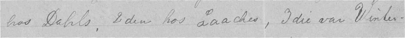hos Dahls, 2den hos Laaches, 3die var Winter-
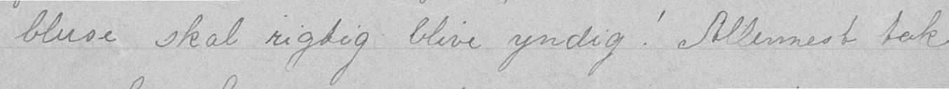bluse skal rigtig blive yndig! Allermest tak
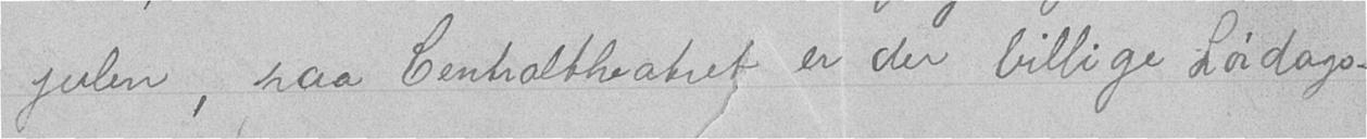julen, paa Centraltheatret er der billige Lørdags-
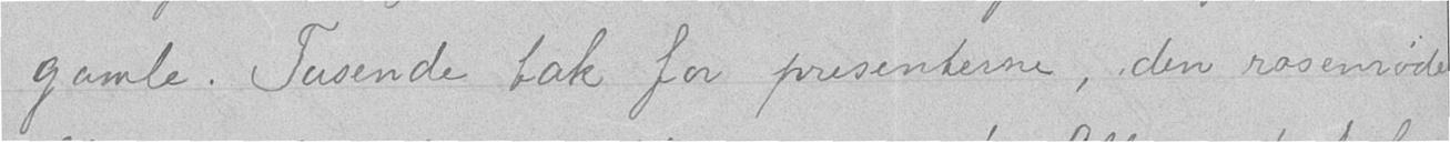gamle. Tusende tak for presenterne, den rosenrøde
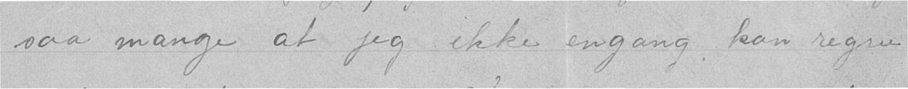saa mange at jeg ikke engang kan regne
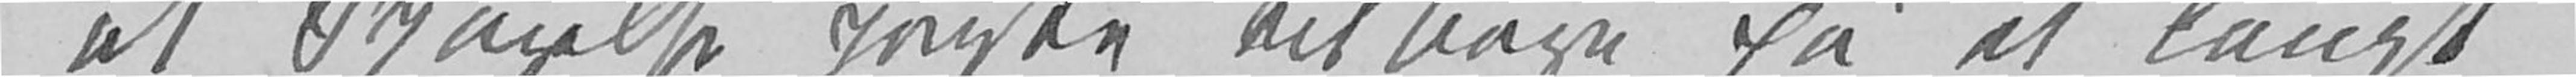et Spøgelse pegte tilbage på et langt
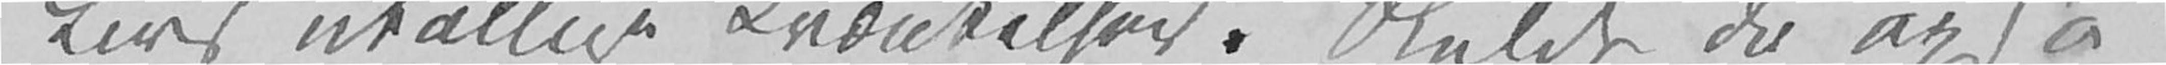Livs utallige Krænkelser. Skulde de også
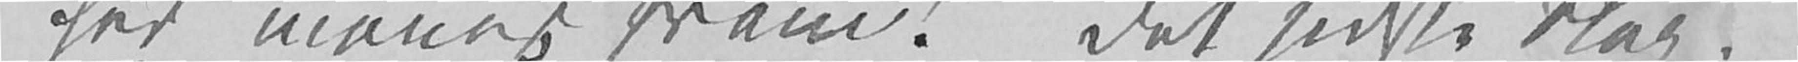her manes frem! Det sidste Slag!
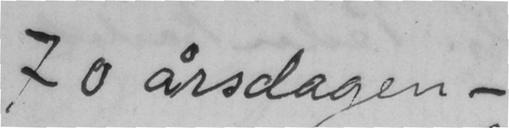70 årsdagen -
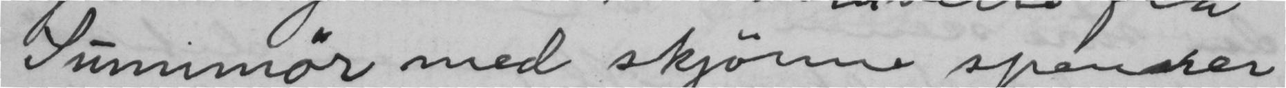Sunnmør med skjønne spenner
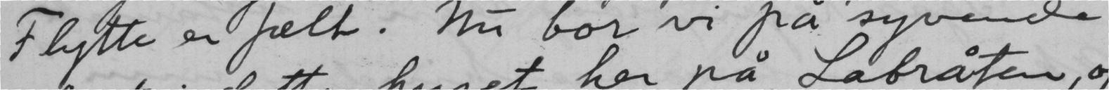Flytte er fælt. Nu bor vi på syvende
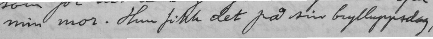min mor. Hun fikk det på sin brylluppsdag,
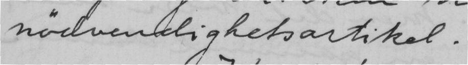nødvendighetsartikel.
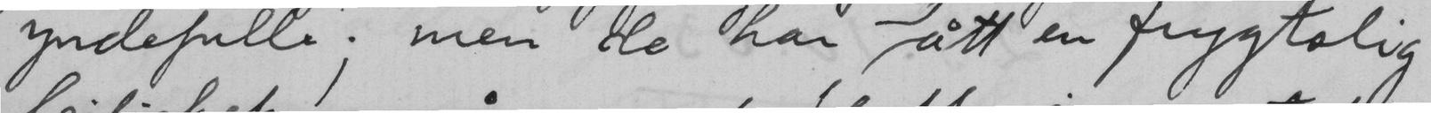yndefulle; men de har fått en frygtelig
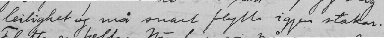leilighet og må snart flytte igjen stakar.
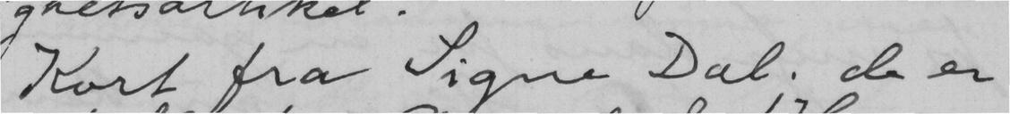Kort fra Signe Dal; de er
